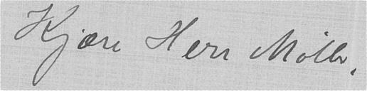Kjære Herr Møller,
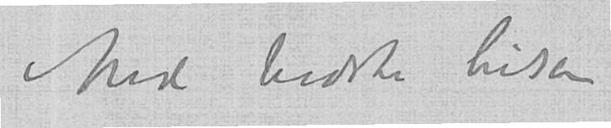Med bedste hilsen
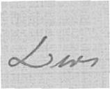Deres
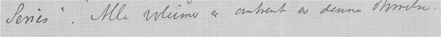Series». Alle volumer er omtrent av denne størrelse.
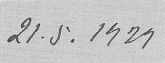21.5.1929
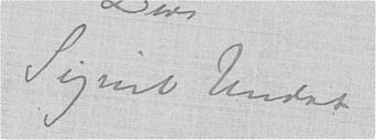Sigrid Undset
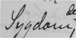Sygdom,
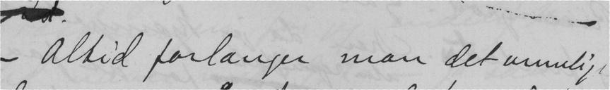– Altid forlanger man det umulige
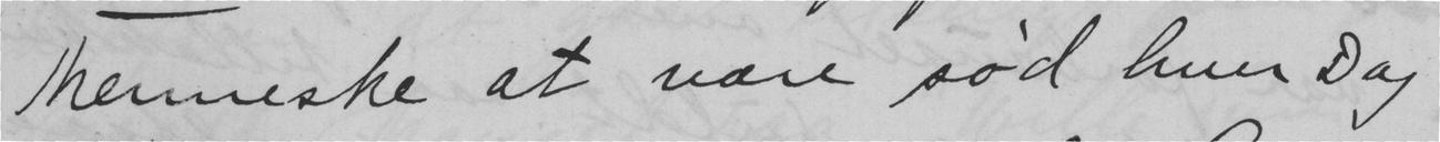menneske at være sød hver Dag
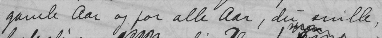gamle aar og for alle Aar, du snille,
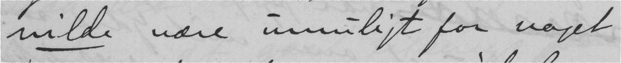vilde være umuligt for noget
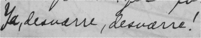Ja, desværre, desværre!
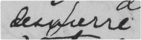desværre
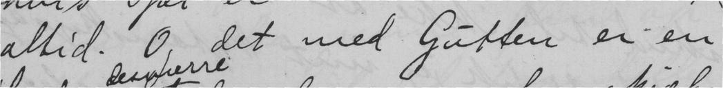altid. Og det med Gutten er en
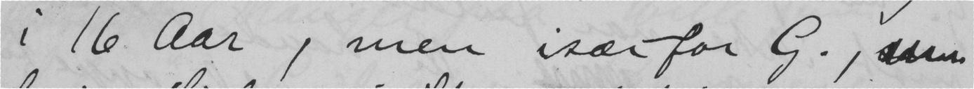i 16. Aar, men især for G.,
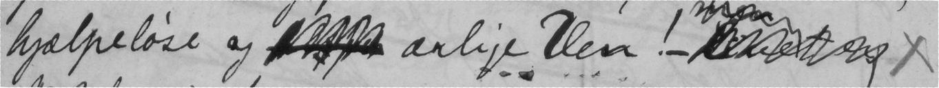hjælpeløse og  ærlige ven!   x
